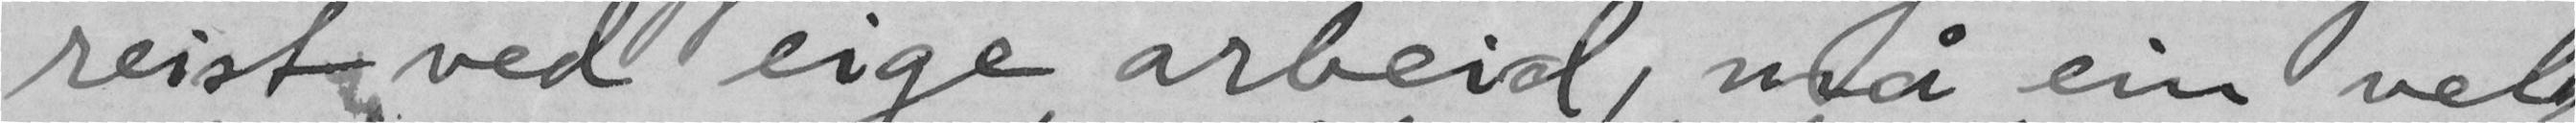reist ved eige arbeid, må ein vel
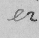er
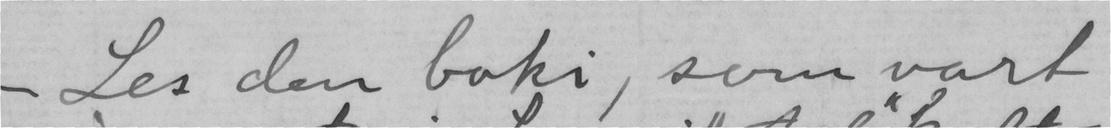- Les den boki, som vart
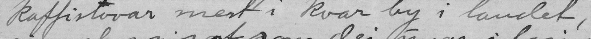kaffistovar mest i kvar by i landet,
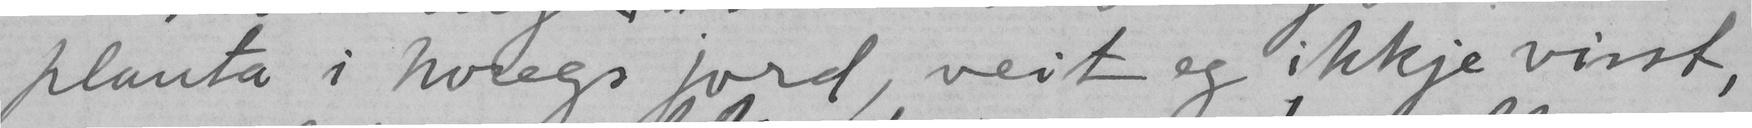planta i Noregs jord, veit eg ikkje visst,
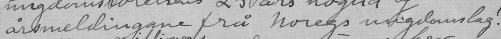årsmeldingane frå Noregs ungdomslag!
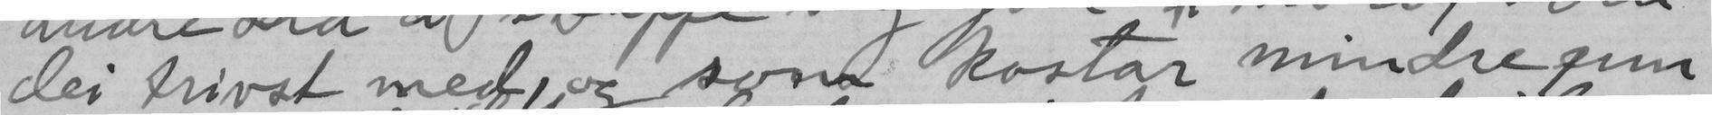dei trivst med,og som kostar mindre enn
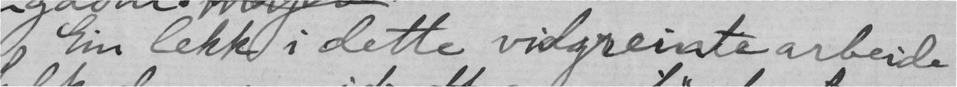Ein lekk i dette vidgreinte arbeide
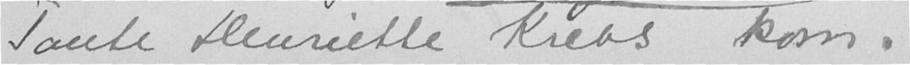Tante Henriette Krebs kom.
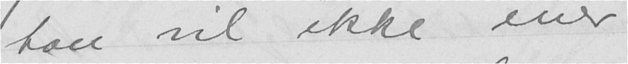han vil ikke mer
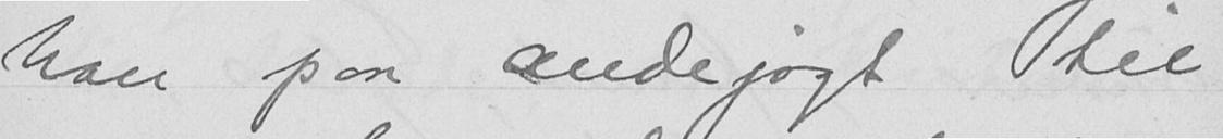han paa andejagt til
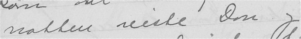natten reiste Don og
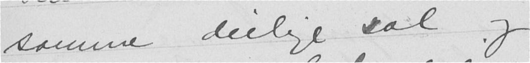samme deilige sol og
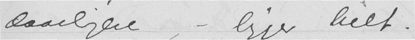daarligere, - ligger helt.
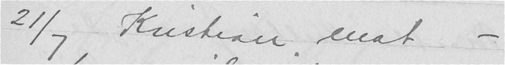21/7 Kristian mat -
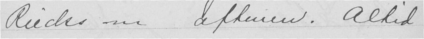Riecks om aftenen. Altid
